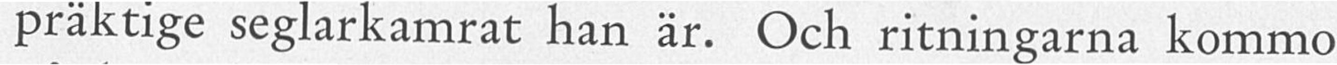praktige seglarkamrat han är. Och ritningarna kommo
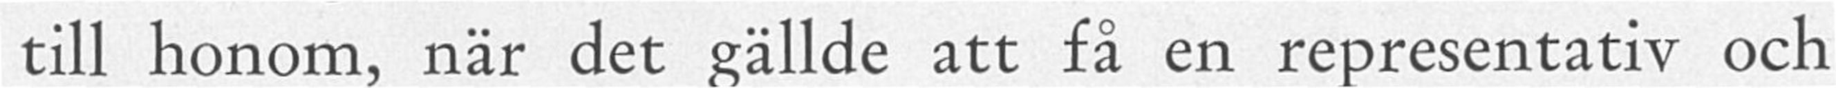till honom, när det gällde att få en representativ och
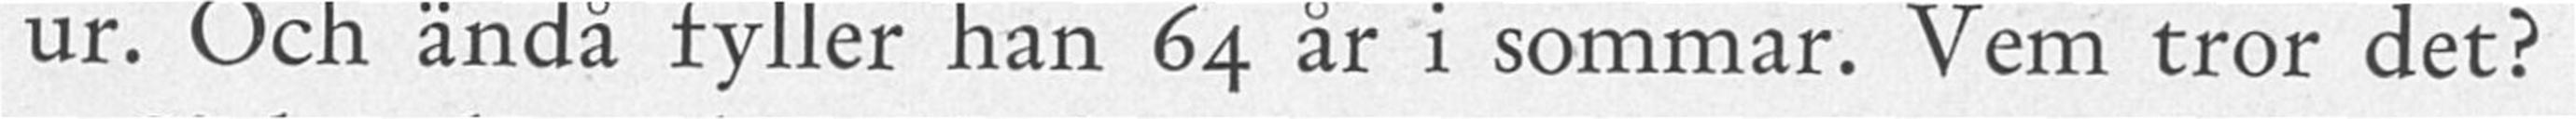ur. Och ändà fyller han 64 år i sommar. Vem tror det?
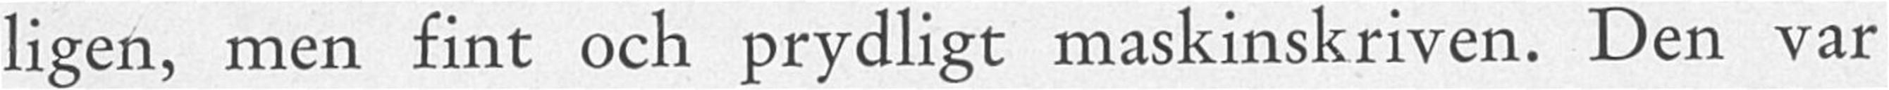ligen, men fint och prydligt maskinskriven. Den var
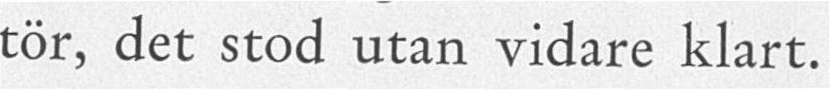tor, det stod utan vidare klart.
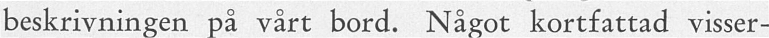beskrivningen på vårt bord. Något kortfattad visser-
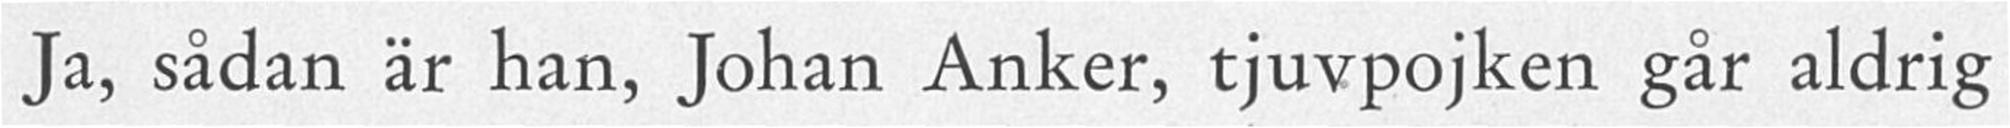Ja, sädan är han, Johan Anker, tjuvpojken går aldrig
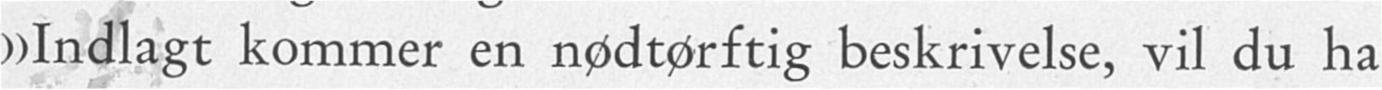"Indlagt kommer en nødtørftig beskrivelse, vil du ha
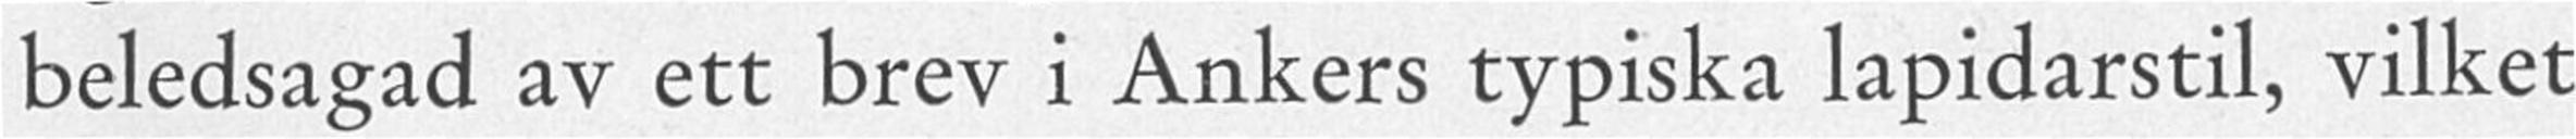beledsagad av ett brev i Ankers typiska lapidarstil, vilket
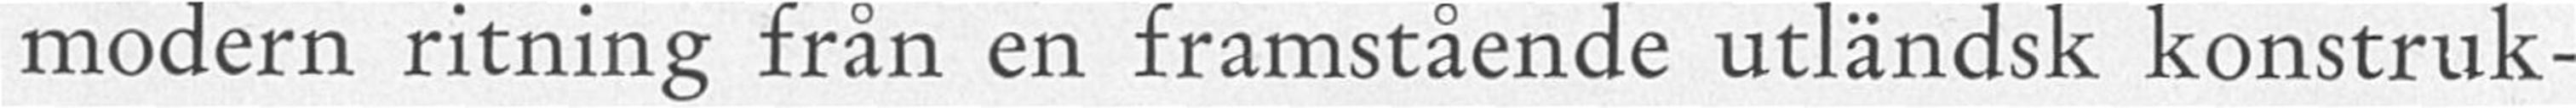modern ritning från en framstående utländsk konstruk-
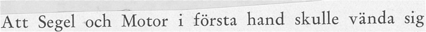Att Segel och Motor i första hand skulle vända sig
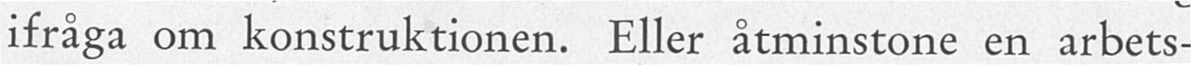ifraga om konstruktionen. Eller åtminstone en arbets-
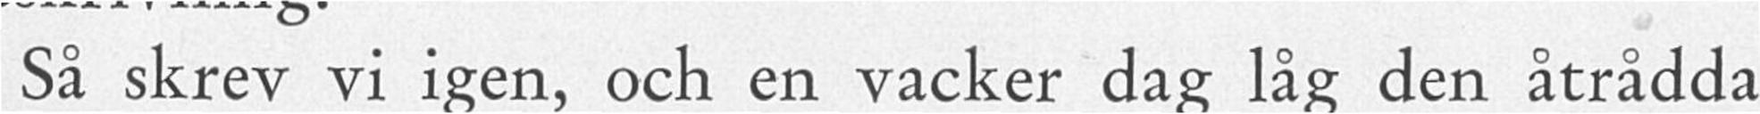Så skrev vi igen, och en vacker dag låg den åtrådda
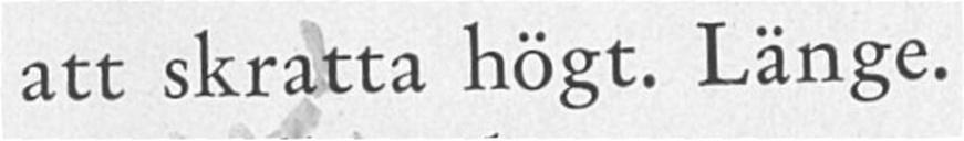att skratta högt. Länge.
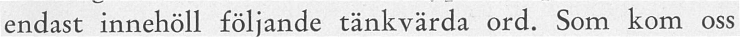endast innehöll följande tänkvärda ord. Som kom oss
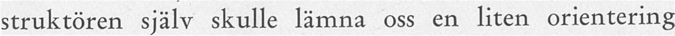struktören själv skulle lämna oss en liten orientering
